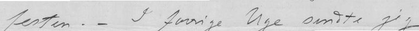festen. - I forrige Uge sendte jeg
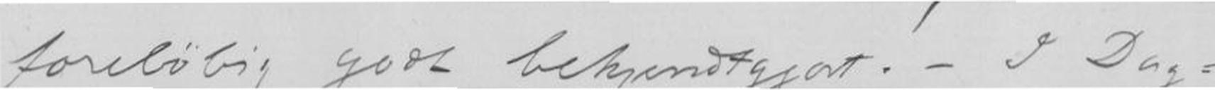foreløbig godt bekjendtgjort! - I Dag-
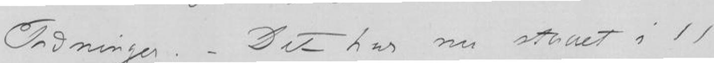Tidninger. - Det har nu staaet i 11
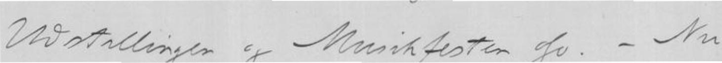Udstillingen og Musikfesten osv. - Nu
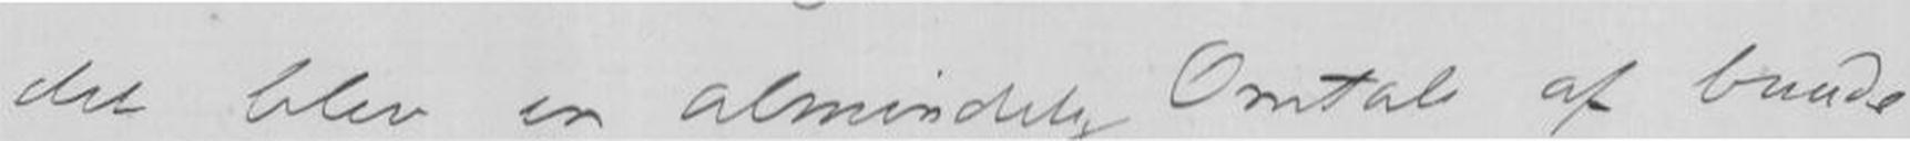det blev en almindelig Omtale af baade
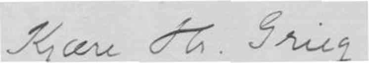Kjære Hr. Grieg.
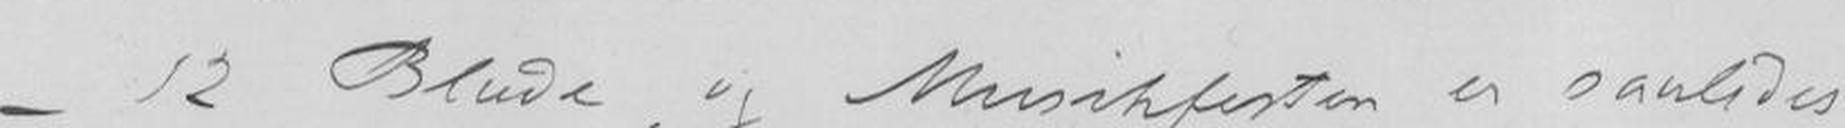-12 Blade, og Musikfesten er saaledes
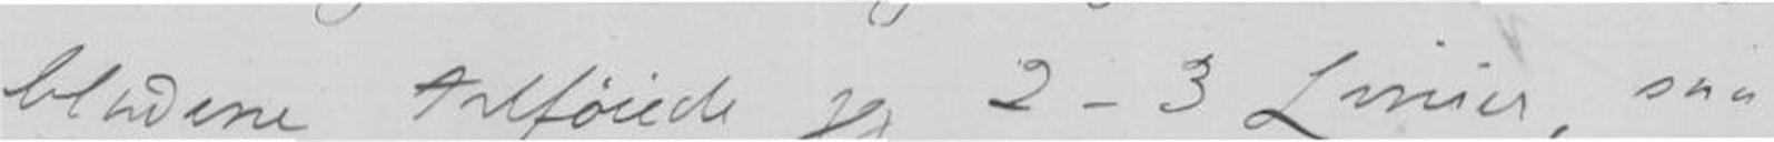bladerne tilføiede jeg 2-3 Linier, saa
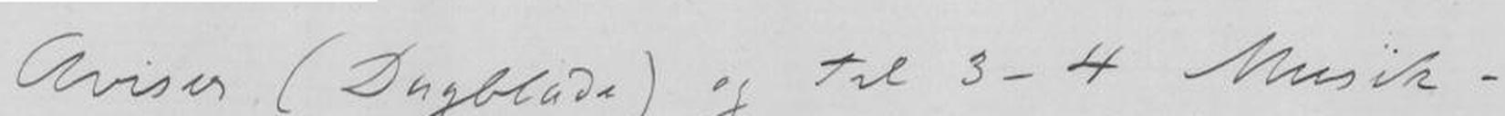Aviser (Dagblade) og til 3-4 Musik-
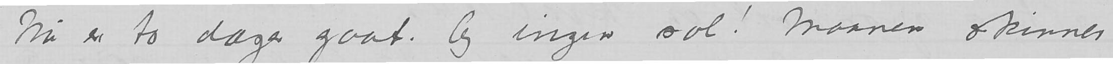Nu er to dager gaat. Og ingen sol! Maanen skinner
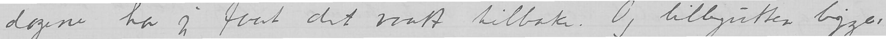dagene har jeg faat det vaatt tilbake. Og lillegutten ligger
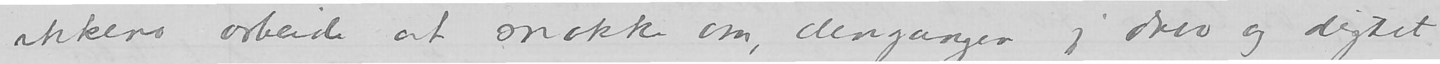ikkeno arbeide at snakke om, dengangen jeg skrev og digtet –.
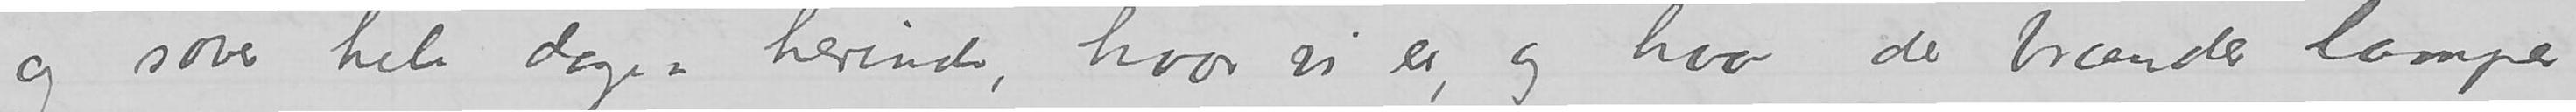og sover hele dagen herinde, hvor vi er, og hvor der brænder lamper
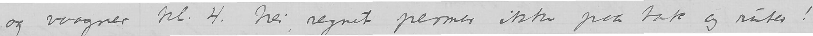og vaagner kl. 4. Nei, regnet permer ikke paa tak og ruter!
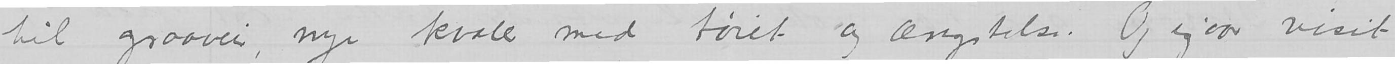til graaveir, nye kvaler med tøiet og ængstelse. Og igaar visit
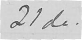21de.
